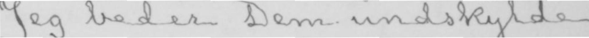Jeg beder Dem undskylde
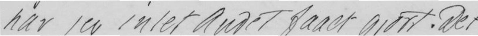har jeg intet Andet faaet gjort. Det
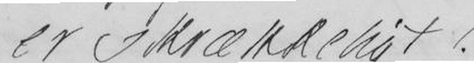er skrækkeligt!
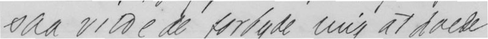saa vilde de forbyde mig at
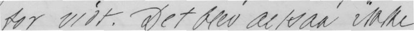for vidt. Det blev altsaa ikke
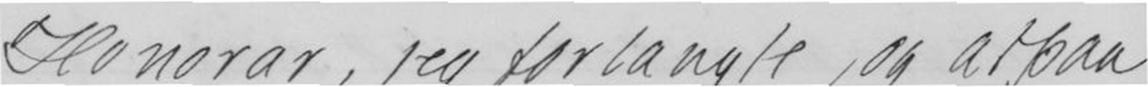Honorat, jeg forlangte, og atpaa
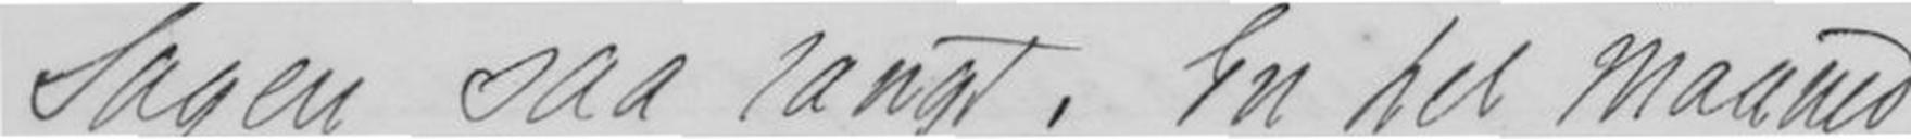Sagen saa langt. En hel maaned
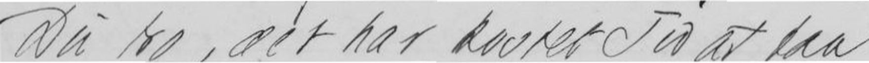Du tro, det har kostet Tid at faa
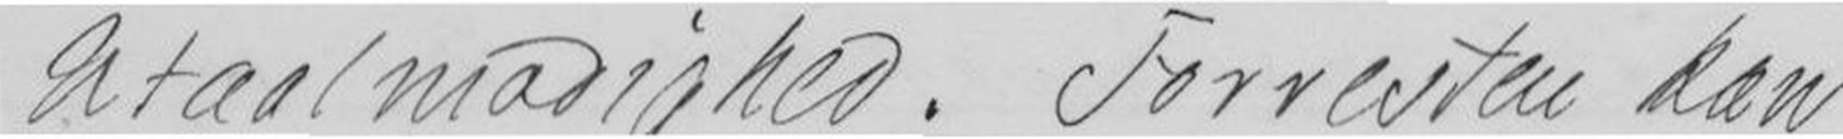Utaalmodighed. Forresten kan
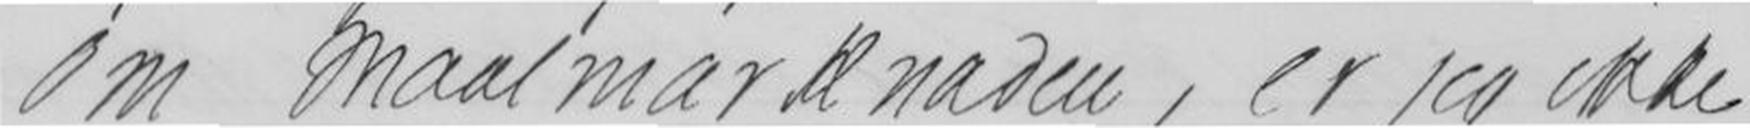om maalmarknaden, er jeg ikke
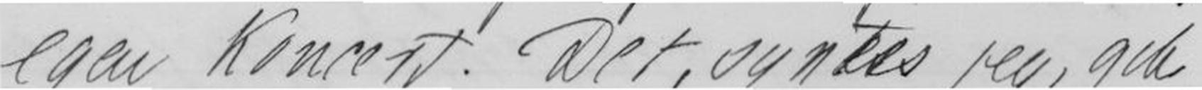egen Koncert. Det, syntes jeg, gik
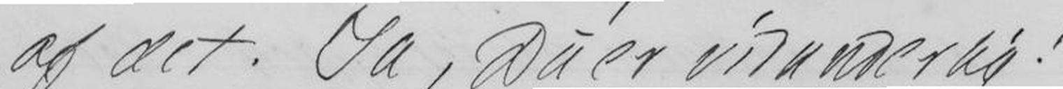af det. ja, Du er vidunderlig!
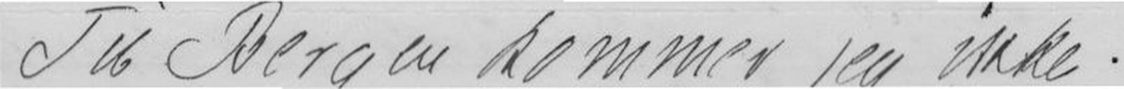Til Bergen kommer jeg ikke
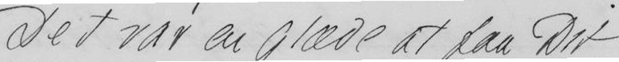Det var en glæde at faa Dit
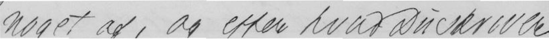noget af, og efter hvad Du skriver
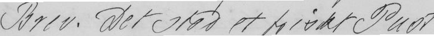Brev. Det stod et friskt Pust
